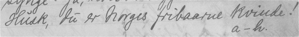Husk, du er Norges "fribaarne kvinde!
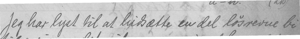Jeg har lyst til at hidsætte en del løsrevne bi-
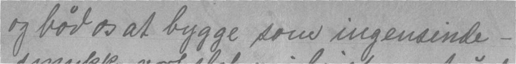og bød os at bygge som ingensinde -
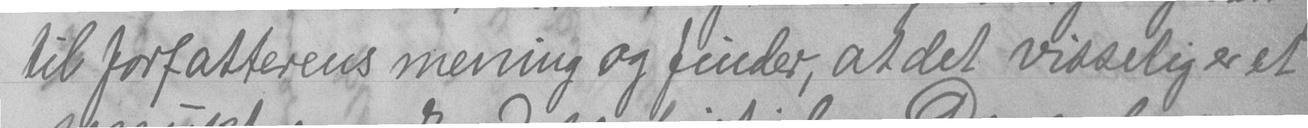til forfatterens mening og finder, at det visselig er et
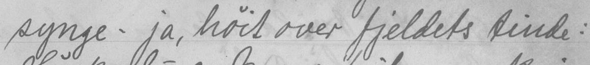synge. ja, høit over fjeldets tinde:
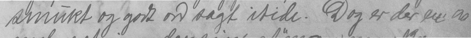smukt og godt ord sagt itide. Dog er der en ga-
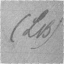(Lvs)
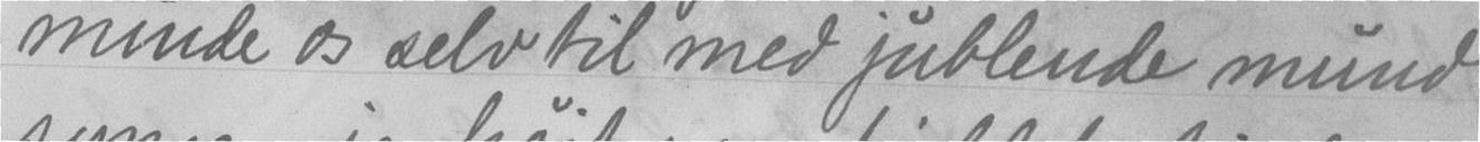minde os selv til med jublende mund
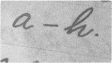a - h.
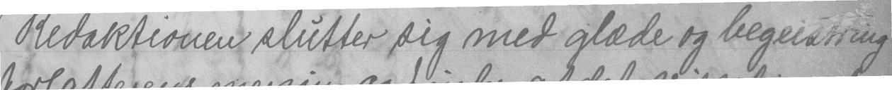Redaktionen slutter sig med glæde og begeistring
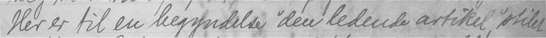Her er til en begyndelse "den ledende artikel," stilet
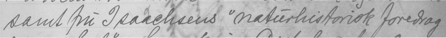samt fru Isachsens "naturhistorisk foredrag
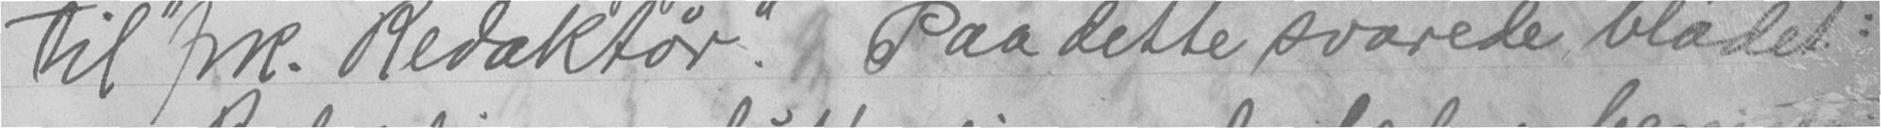til "frk. Redaktør."  Saa dette svarede bladet:
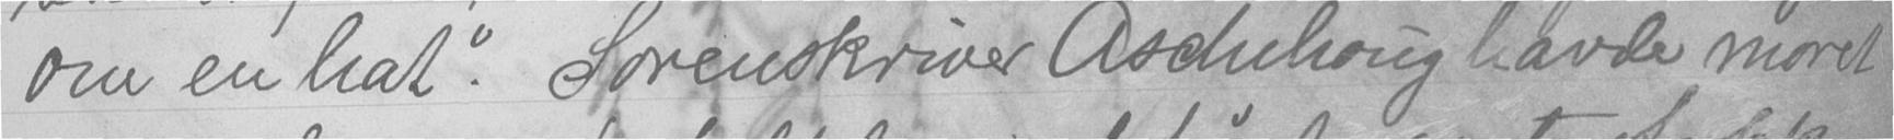om en hat". Sorenskriver Aschehong havde moret
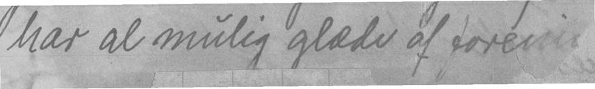har al mulig glæde af forenin
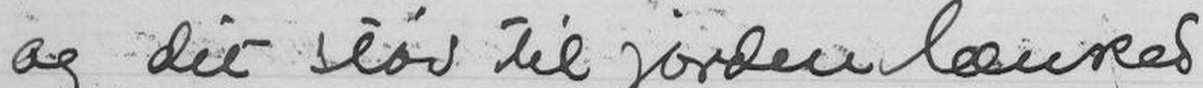og dit støv til jorden lænked
